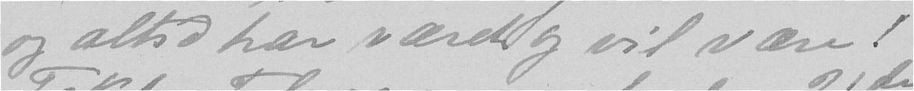og altid har været og vil være!
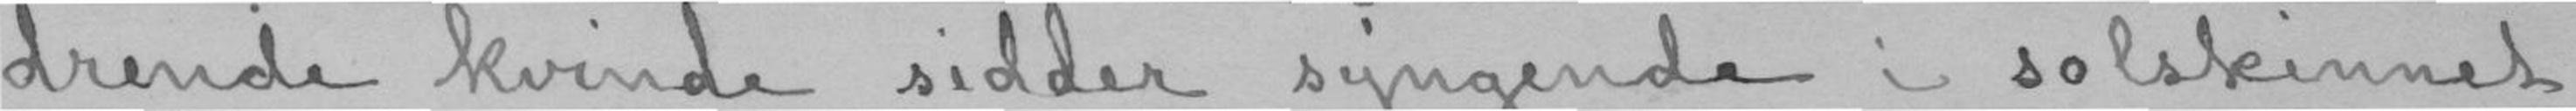drende kvinde sidder syngende i solskinnet
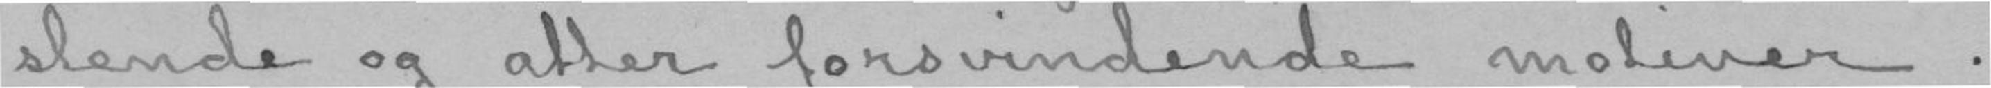slende og atter forsvindende motiver.
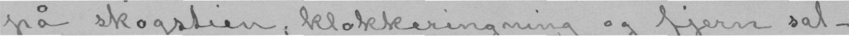på skogstien; klokkeringning og fjern sal-
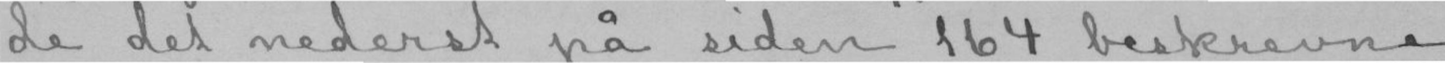de det nederst på siden 164 beskrevne
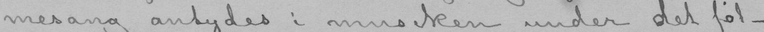mesang antydes i musiken under det føl-
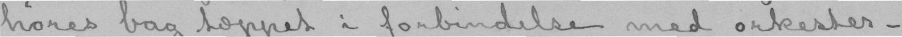høres bag tæppet i forbindelse med orkester-
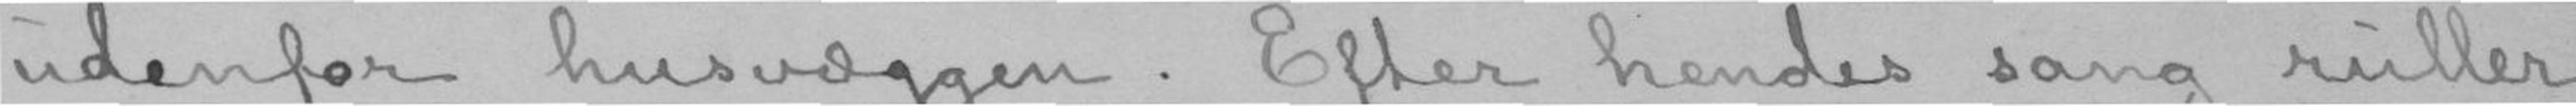udenfor husvæggen. Efter hendes sang ruller
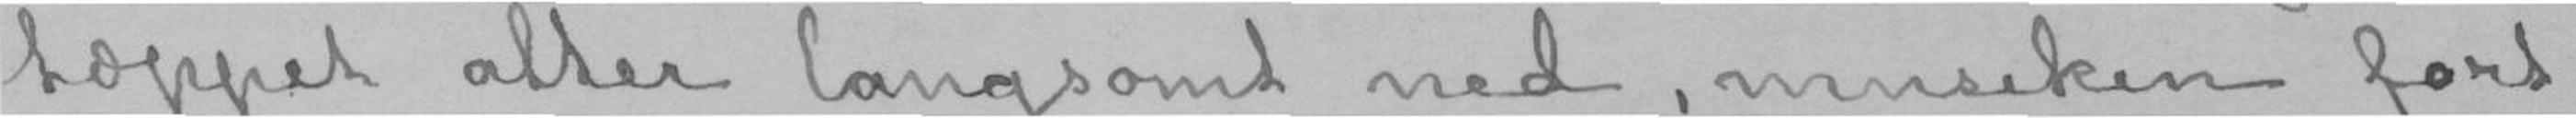tæppet atter langsomt ned, musiken fort-
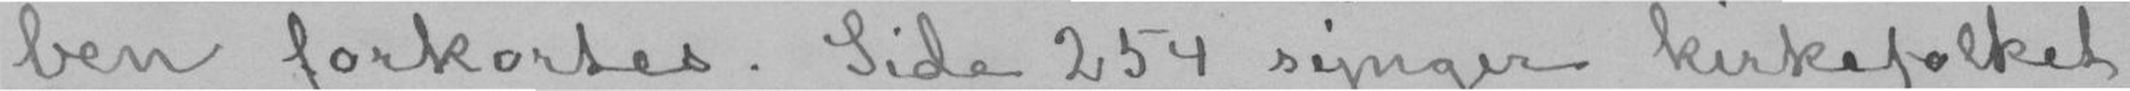ben forkortes. Side 254 synger kirkefolket
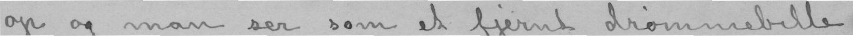op og man ser som et fjernt drømmebille-
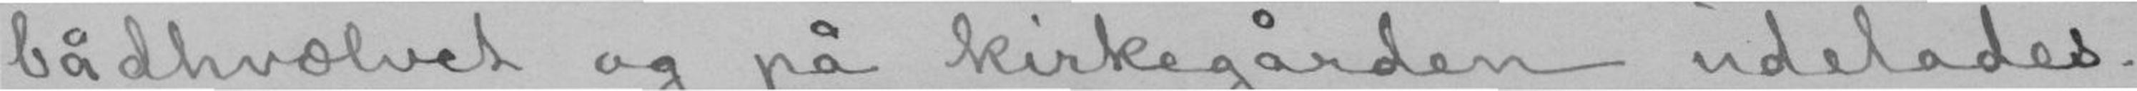bådhvælvet og på kirkegården udelades.
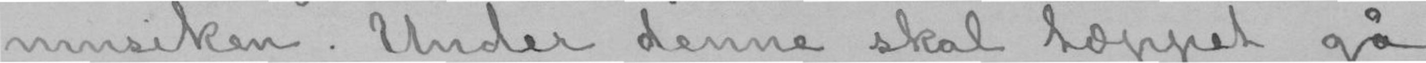musiken. Under denne skal tæppet gå
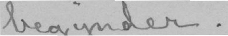begynder.
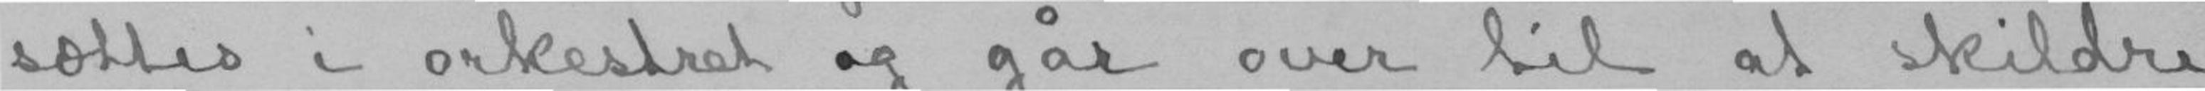sættes i orkestret og går over til at skildre
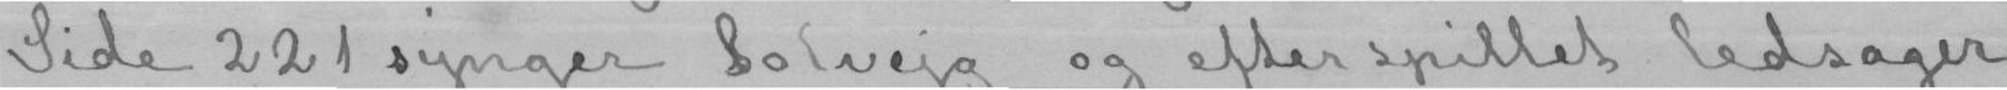Side 221 synger Solvejg og efter spillet ledsager
